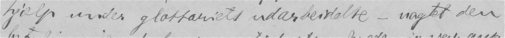hjælp under glossariets udarbeidelse - uagtet den
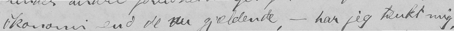økonomi end de nu gjældende, - har jeg tænkt mig,
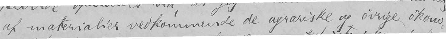af materialier vedkommende de agrariske og øvrige økono-
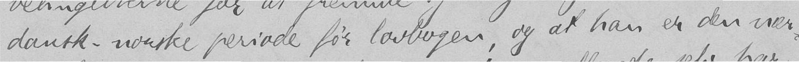dansk-norske periode før lovbogen, og at han er den nær-
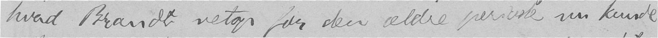hvad Brandt netop for den ældre periode nu kunde
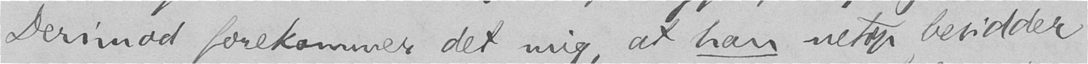Derimod forekommer det mig, at han netop besidder
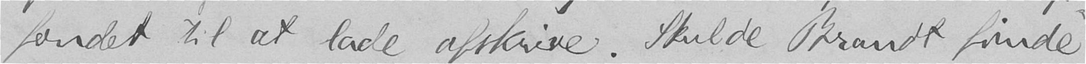fondet til at lade afskrive. Skulde Brandt finde
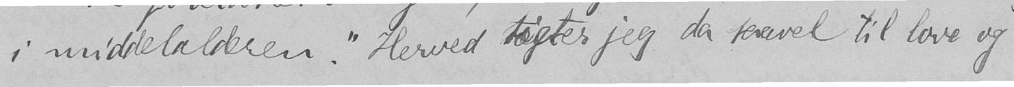i middelalderen." Herved sigter jeg da saavel til love og
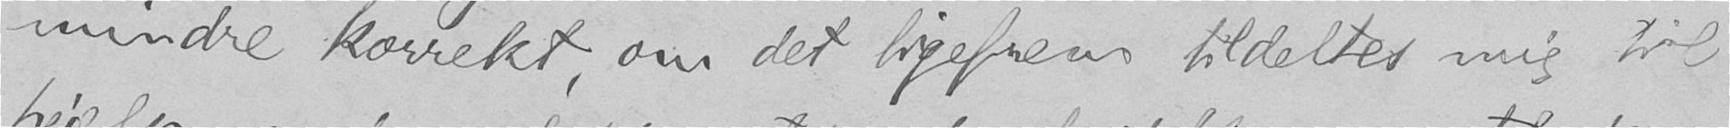mindre korrekt, om det ligefrem tildeltes mig til
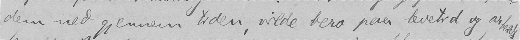dem ned gjennem tiden, vilde bero paa levetid og arbeids-
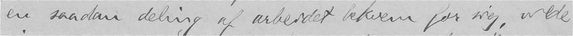en saadan deling af arbeidet bekvem for sig, vilde
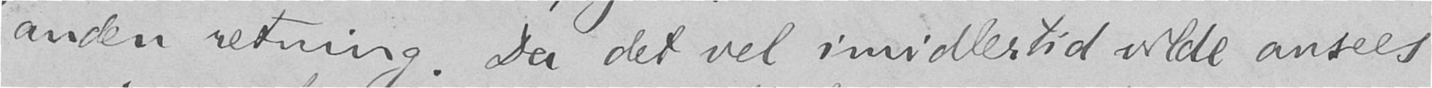anden retning. Da det vel imidlertid vilde ansees
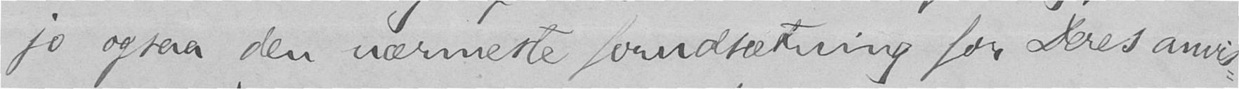jo ogsaa den nærmeste forudsætning for Deres anvis-
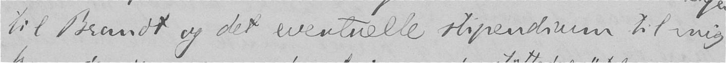til Brandt og det eventuelle stipendium til mig
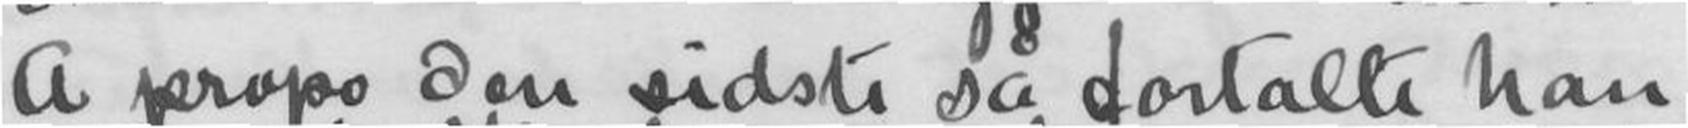A propo den sidste så fortalte han
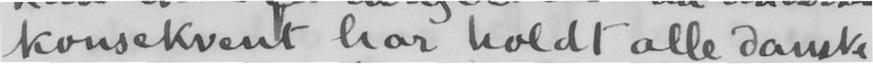konsekvent har holdt alle danske
